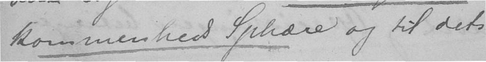kommenheds Sphære og til dets
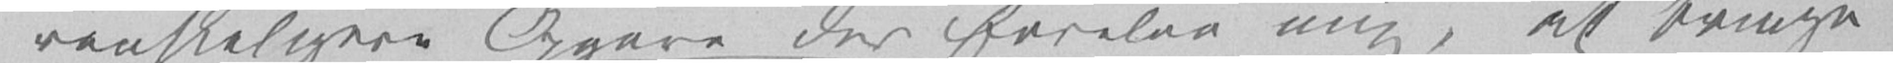vanskeligere Opgave der forelaa mig, at bringe
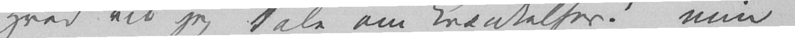Hvad vil jeg tale om Krænkelser! min
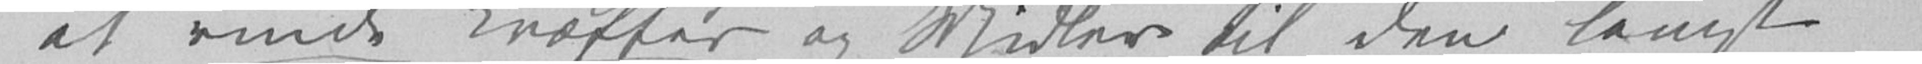at vinde Kræfter og Midler til den langt
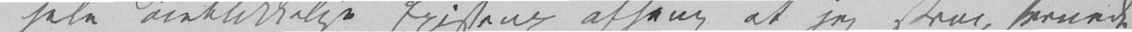hele øieblikkelige Existenz afhang [af] at jeg strax hernede
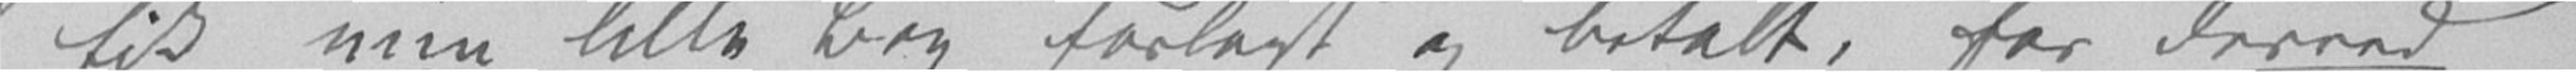fik min lille Bog forlagt og betalt, for derved
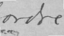fordre
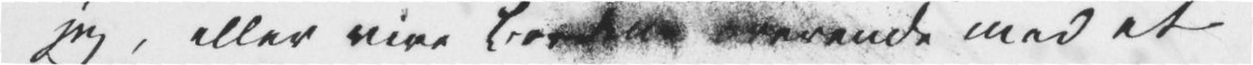jeg, eller rive Bordet overende med et
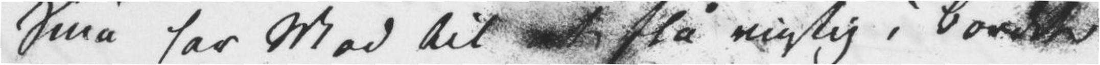Små har Mod til at slå rigtig i Bordet
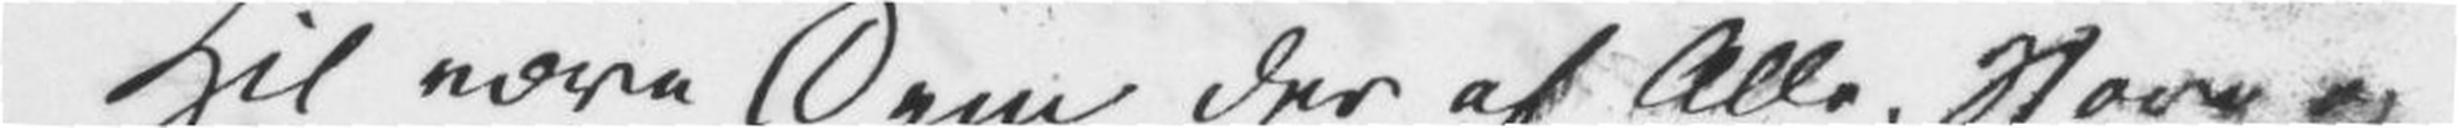Hil være Dem der af Alle, Store og
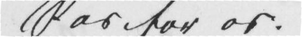Pas for os.
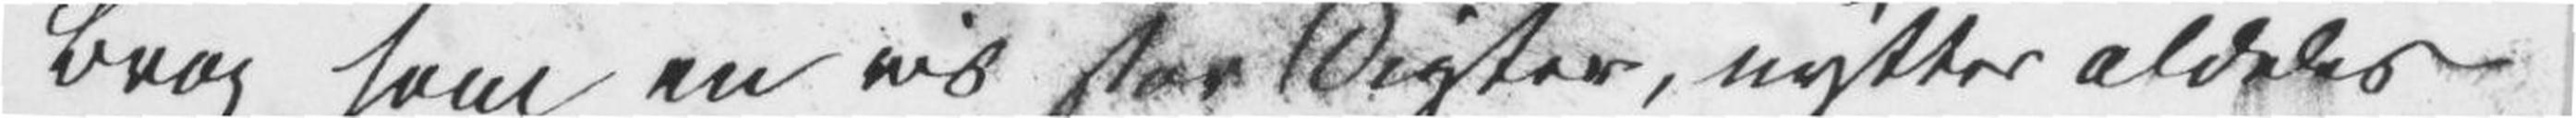Brag som en vis stor Digter, nytter aldeles
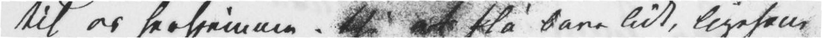til os herhjemme, thi at slå bare lidt, ligesom
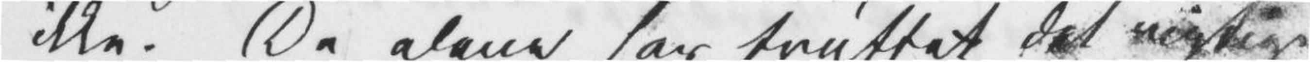ikke. De alene har truffet det rigtige
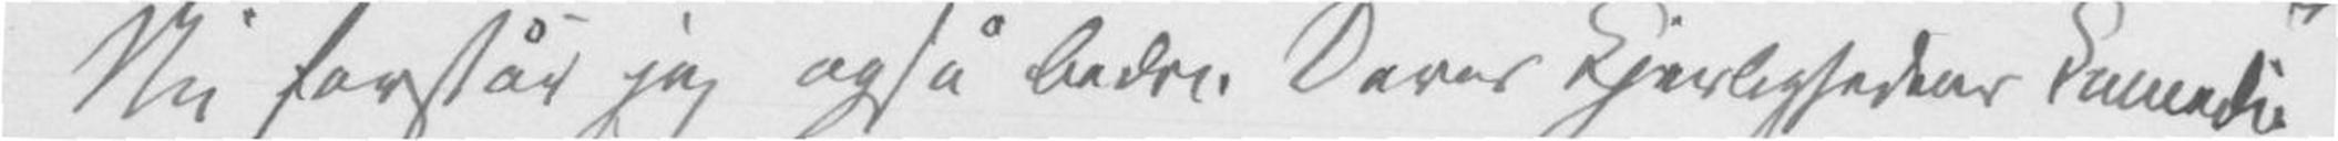Nu forstår jeg også bedre Deres «Kjerlighedens Komedie»
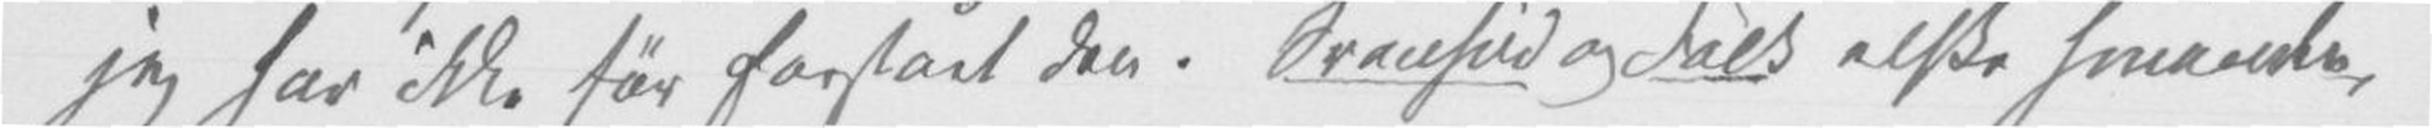jeg har ikke før forstået den. Svanhild og Falk elske hinanden,
